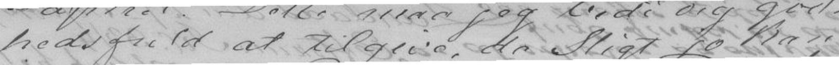hedsfuld at tilgive, da sligt jo kan
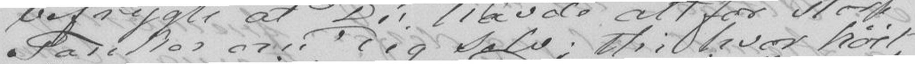tanker om Dig selv; thi hvor høit
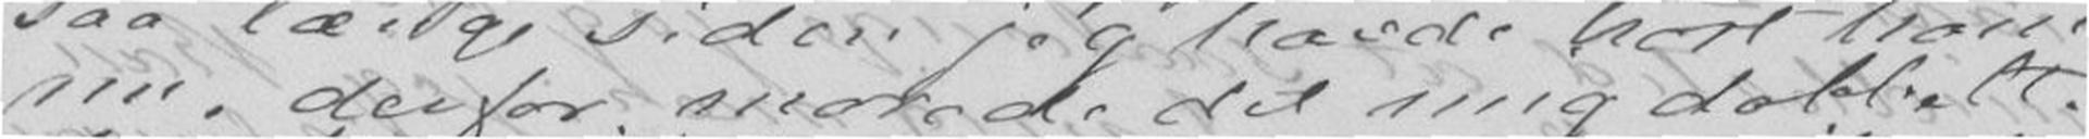nu, derfor morede det mig dobbelt.
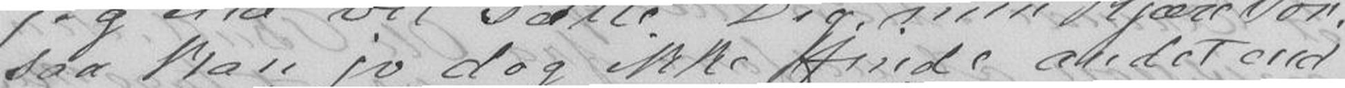saa kan jo dog ikke finde andet end
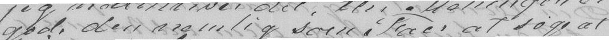god, den nemlig som Faer at søge at
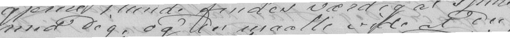med Dig, og Du maatte vide at Du
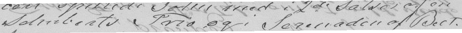Schuberts Trio og i Serenaden af Beet-
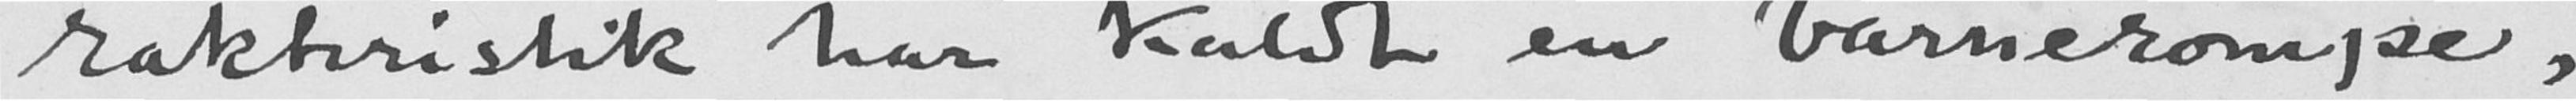rakteristik har kaldt en "barnerompe",
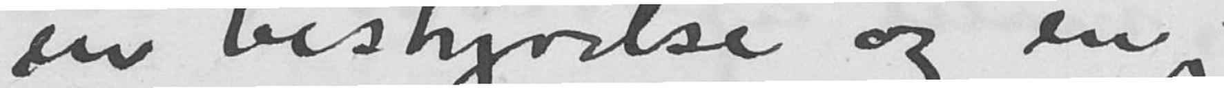en bestyrelse og en
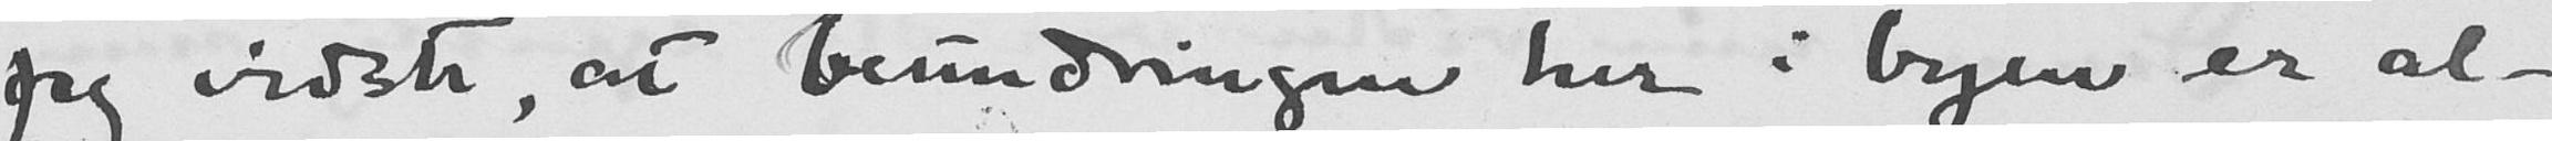jeg vidste, at beundringen her i byen er al-
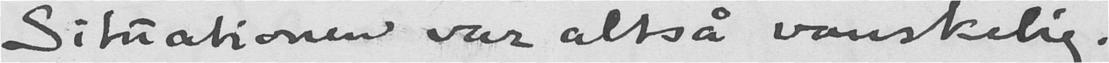Situationen var altså vanskelig.
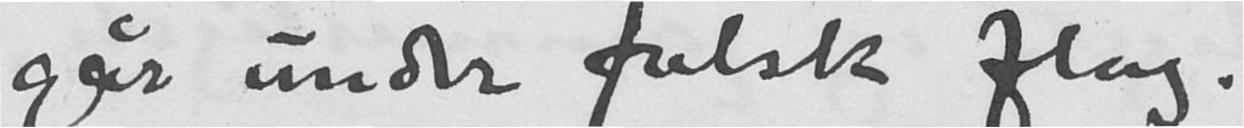går under falsk flag.
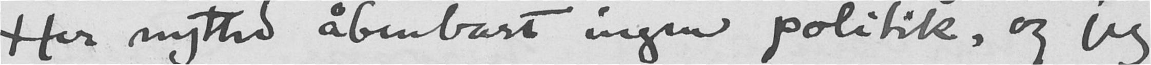Her nytted åbenbart ingen politik, og jeg
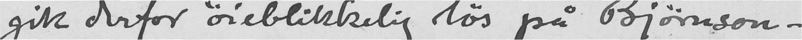gik derfor øieblikkelig løs på Bjørnson-
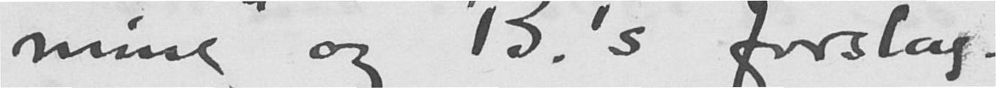ning" og B.'s forslag.
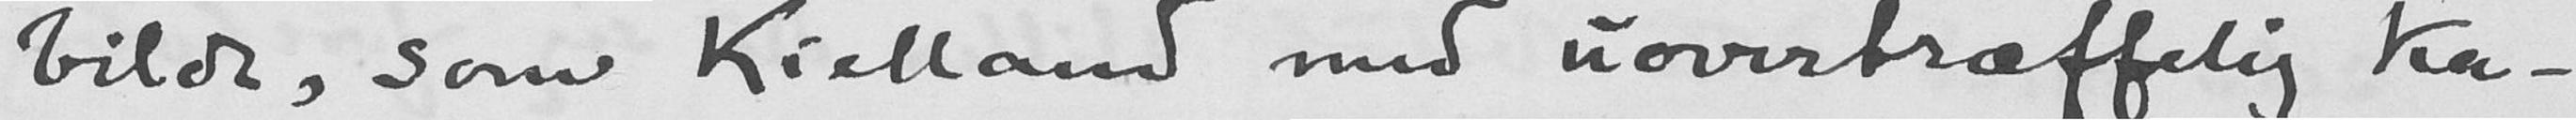bilde, som Kielland med uovertræffelig ka-
